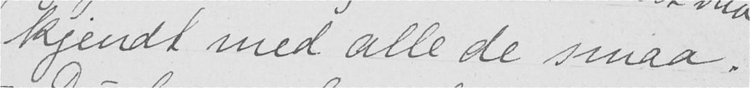kjendt med alle de smaa.
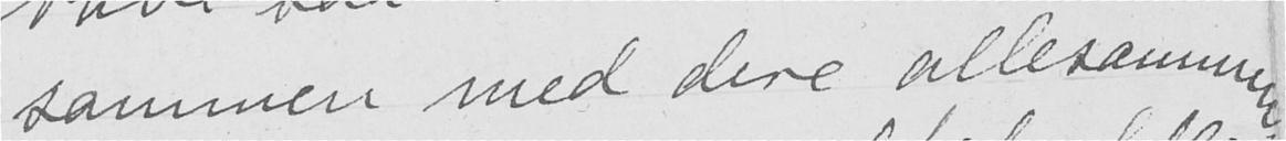sammen med dere allesammen.
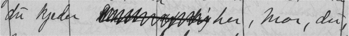du kjeder  her, Mor, du,
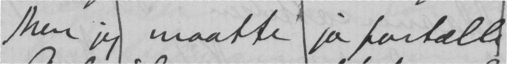Men jeg maatte jo fortælle
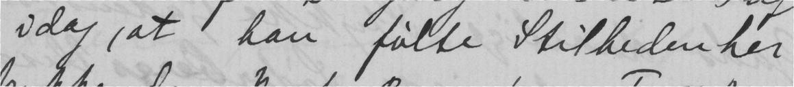idag, at han følte Stilheden her
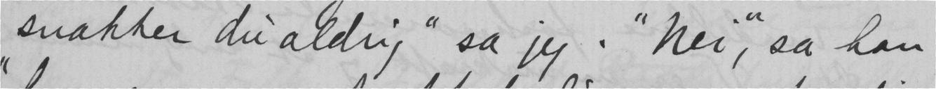snakker du aldrig" sa jeg. "Nei", sa han
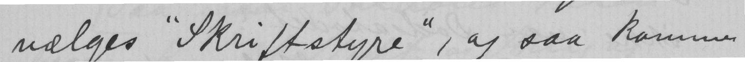vælges "Skriftstyre", og saa kommer
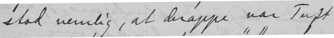stod nemlig, at deroppe var Tuft
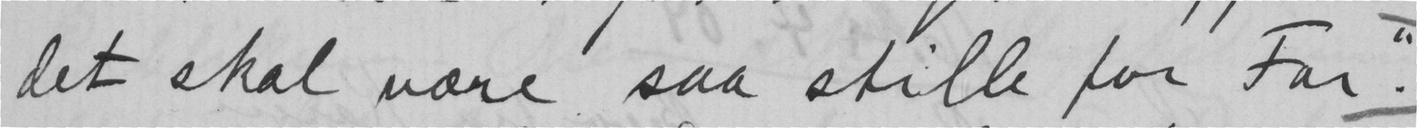det skal være saa stille for Far".
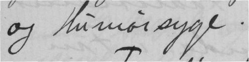og Humørsyge.
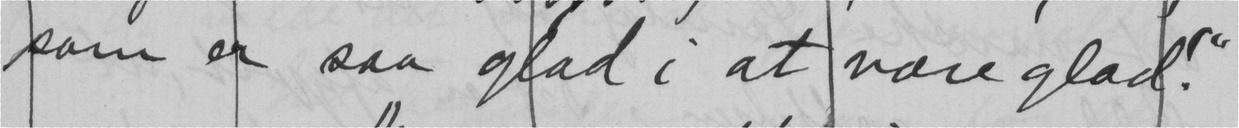som er saa glad i at være glad!"
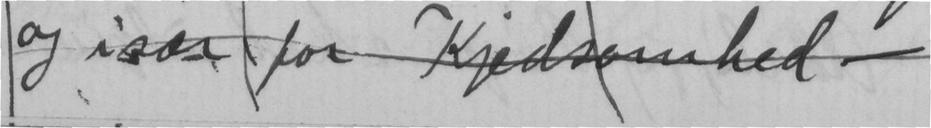og især for Kjedsomhed -
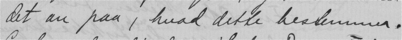det an paa, hvad dette bestemmer.
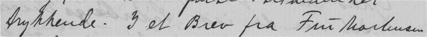trykkende. I et Brev fra Fru Mortensen
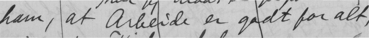ham at Arbeide er godt for alt,
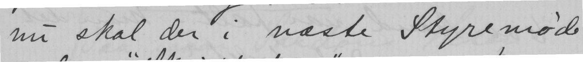nu skal der i næste Styremøde
